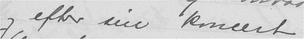og efter sin koncert
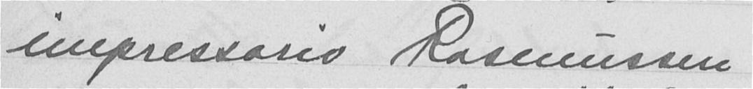impressario Rasmussen
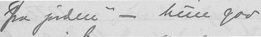fra jorden" - hun gav
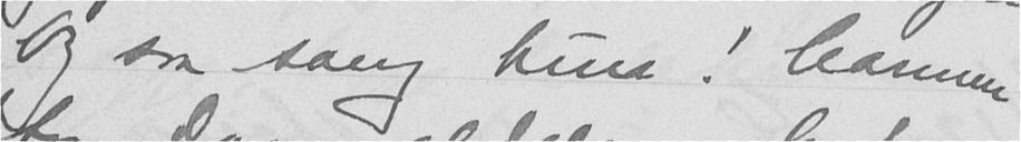Og saa sang hun! Carmen
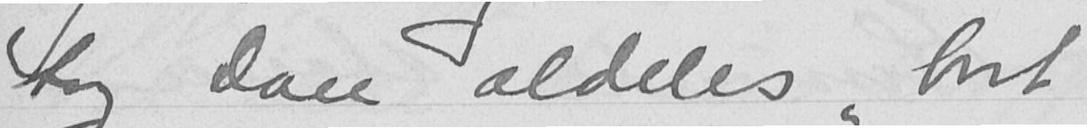tog Don aldeles "bort
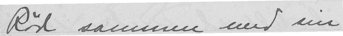Rød sammen med sin
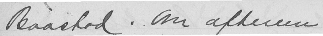Baastad. Om aftenen
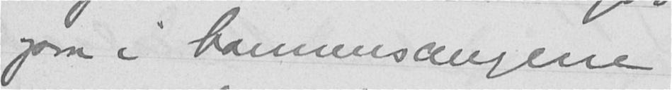ogsaa i Carmensangene
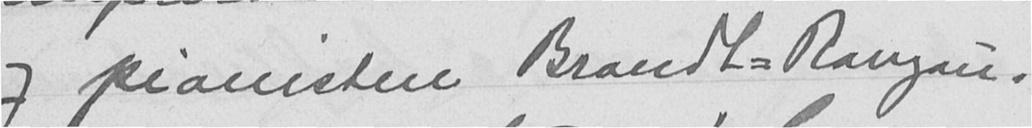og pianisten Brandt-Ranzau.
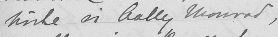hørte vi Cally Monrad,
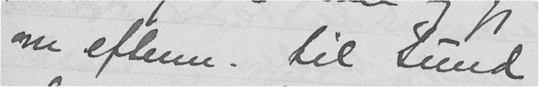om efterm. til Sund
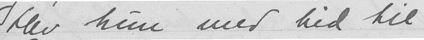blev hun med hid til
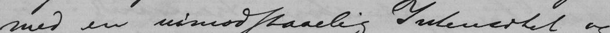med en uimodstaaelig Intensitet og
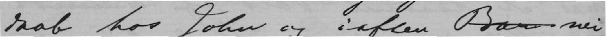daab hos John og iaften Bar nei
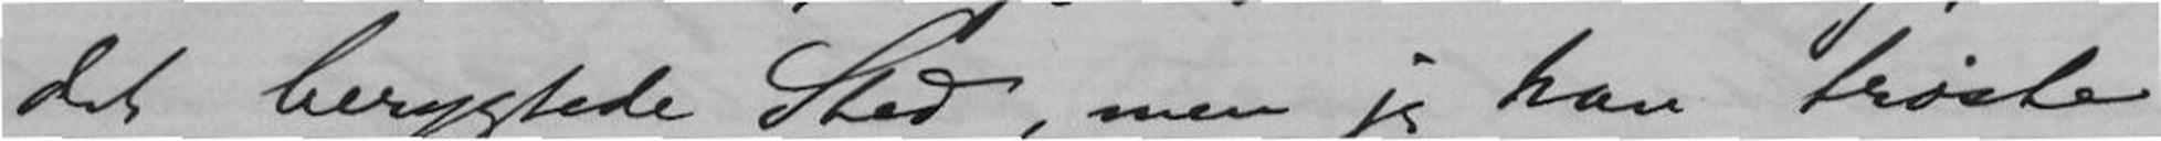det berygtede Sted, men jeg kan trøste
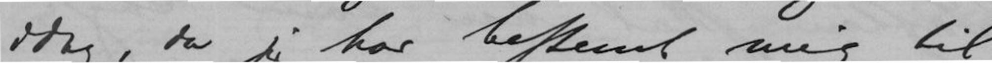idag, da jeg har bestemt mig til
